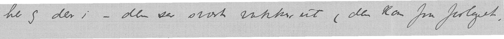her og der i – den ser svært vakker ut (den kom fra forlaget,
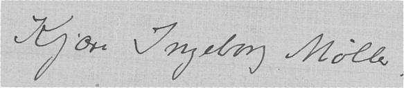Kjære Ingeborg Møller,
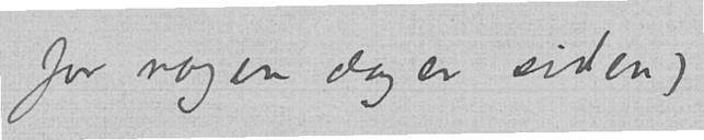for nogen dager siden)
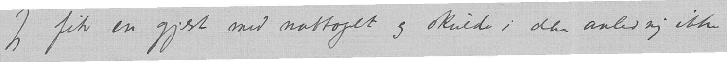Jeg fik en gjest med nattoget og skulde i den anledning ikke
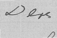Deres
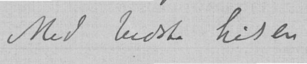Med bedste hilsen
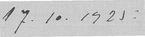17.10.1925.
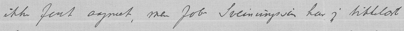ikke faat aapnet, men Job Sveinungsson har jeg tittelæst
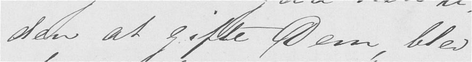den at gifte Dem, blev
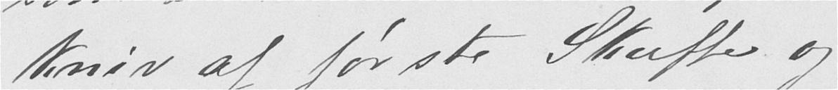kniv af første Skuffe og
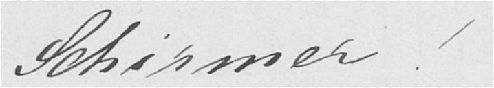Schirmer!
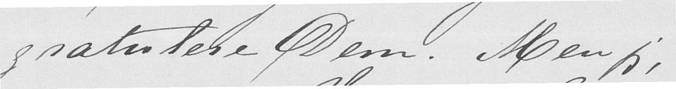gratulere Dem. Men jeg,
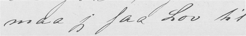maa jeg faa Lov til
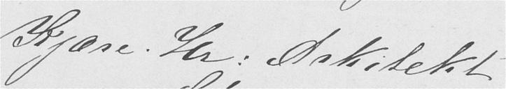Kjære. Hr. Arkitekt
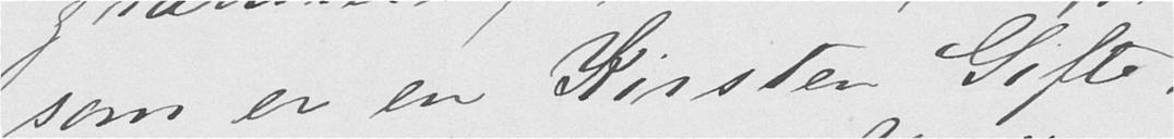som er en Kirsten Gifte-
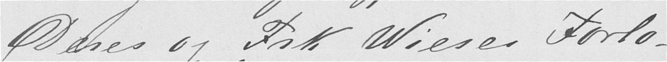Deres og Frk Wieses Forlo-
